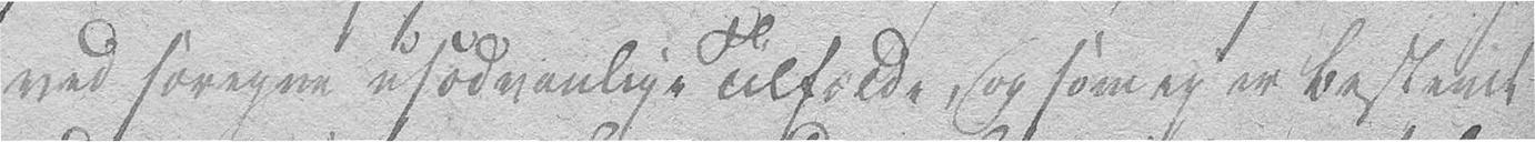ved særegne usædvanlige Tilfælde, og som ey er bestemt
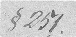§ 251.
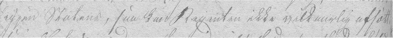igjen Statens, saa kan Regenten ikke vilkaarlig afsætte
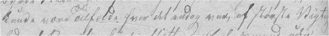kunde være Tilfælde hvor det endog var af største Vigtig-
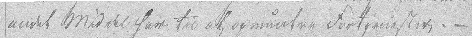andet Middel har til at opmuntre Fortjenester. –
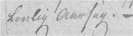lovlig Aarsag. –
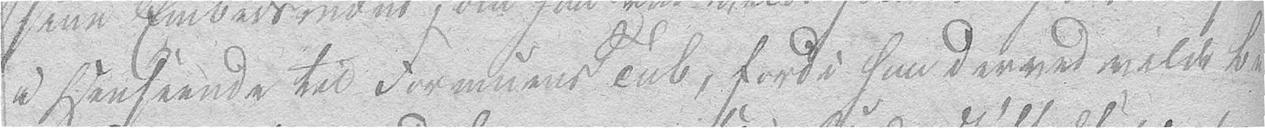i Henseende til Formuens Tab, fordi han derved vilde be-
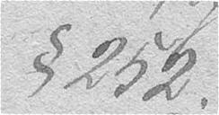§ 252.
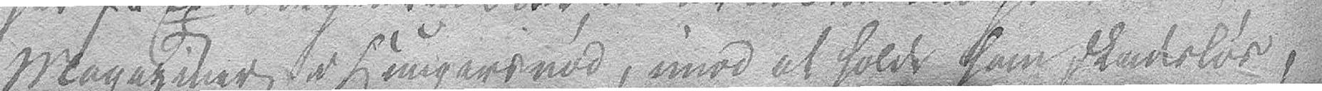Magaziner i en Hungersnød, imod at holde ham skadesløs,
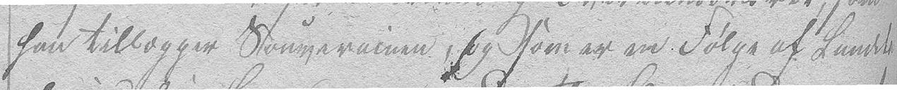han tillægger Souverainen, og som er en Følge af Landets
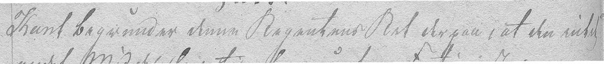Kant begrunder denne Regentens Ret derpaa, at den intet
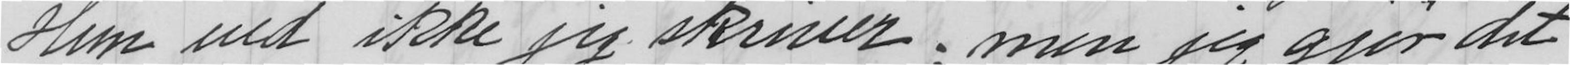Hun ved ikke jeg skriver; men jeg gjør det
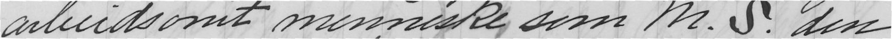arbeidsomt menneske som M. S. den
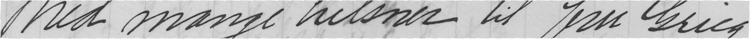Med mange hilsner til fru Grieg
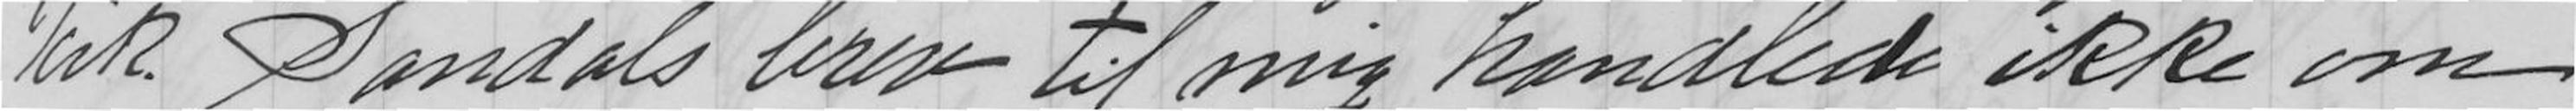Frk. Sandals brev til mig handlede ikke om
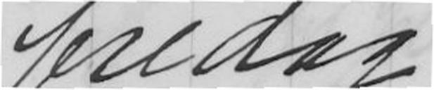fredag
